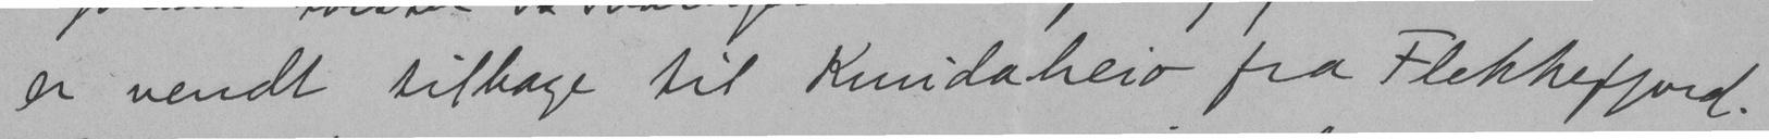er vendt tilbage til Knudaheio fra Flekkefjord.
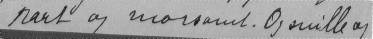rart og morsomt. Og snille og
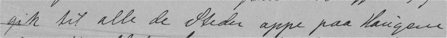gik til alle de Steder oppe paa Haugene
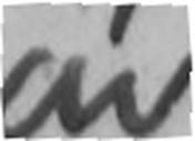av
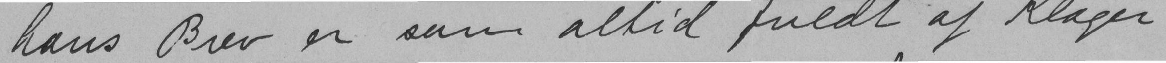hans Brev er som altid fuldt af Klager
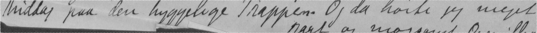Middag paa den hyggelige Trappen. Og da hørte jeg meget
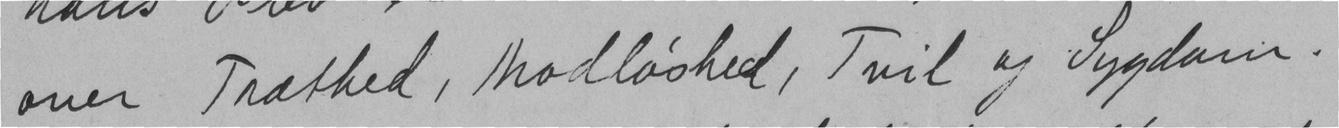over Træthed, Modløshed, Tvil og Sygdom.
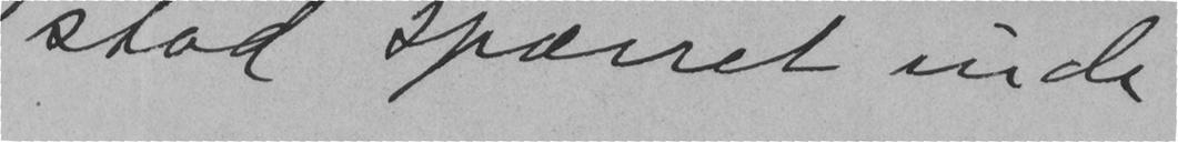stod spærret inde
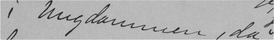i Ungdommen, da
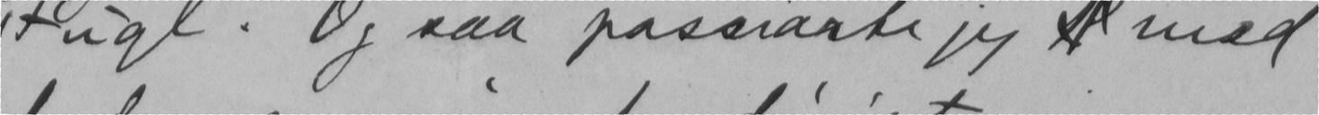Fugl. Og saa passiarte jeg  med
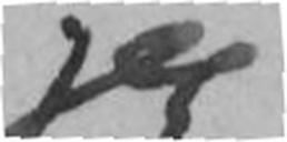jeg
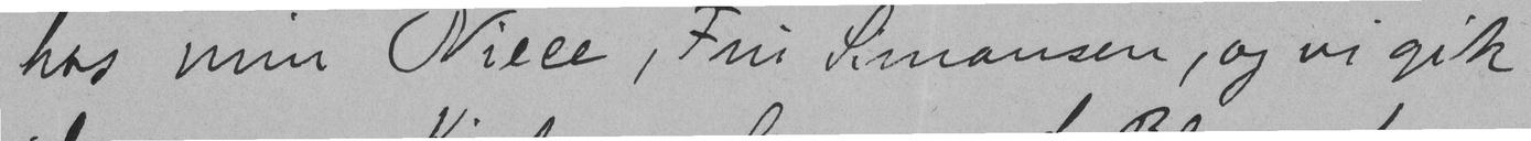hos min Niece, Fru Simonsen, og vi gik
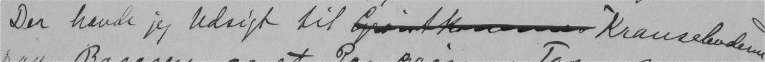Der havde jeg Udsigt til  Kransebodene
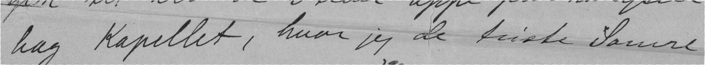bag Kapellet, hvor jeg de triste Somre
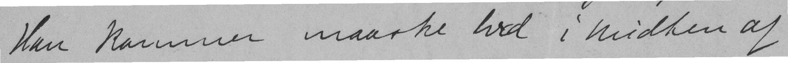Han kommer maaske hid i midten af
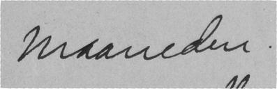Maaneden.
